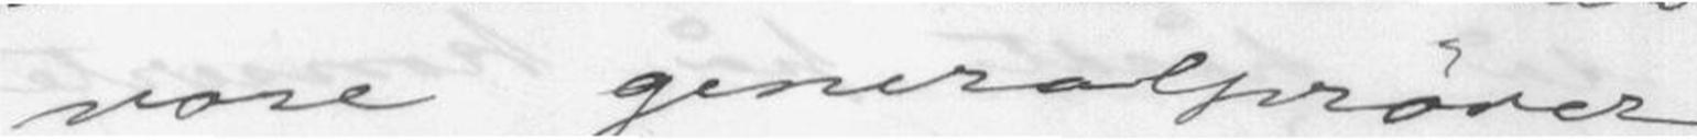vore generalprøver!
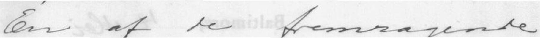En af de fremragende
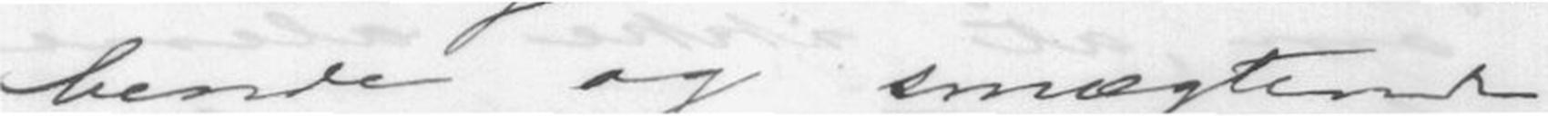bende og smægtende
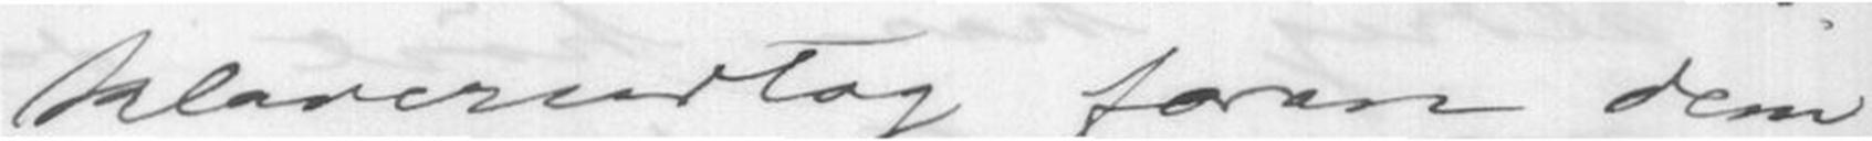klaverudtog forand dem.
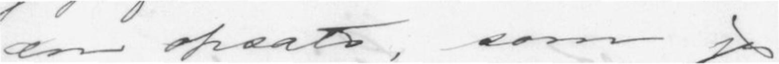en opsats, som jeg
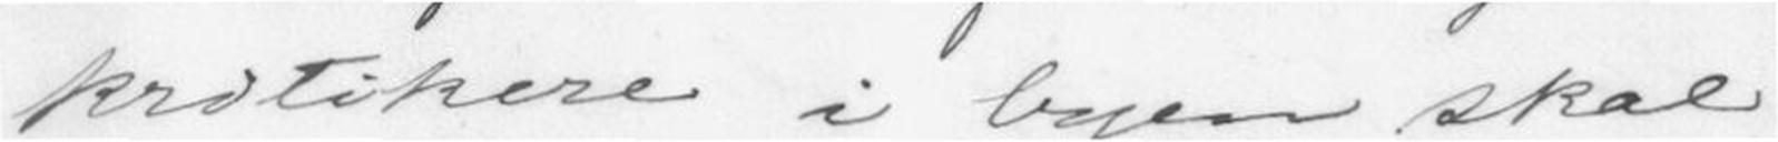kritikere i byen skal
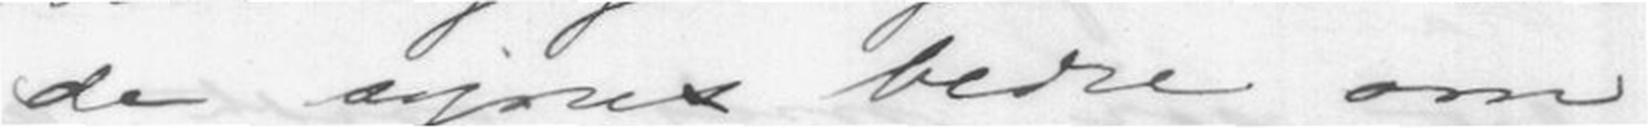de synes bedre om
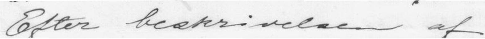Efter beskrivelsen af
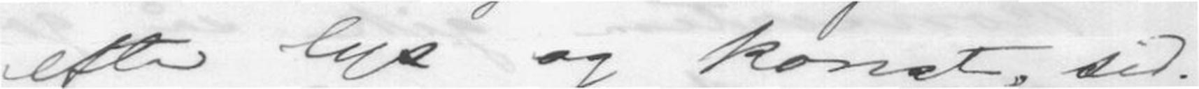efter lys og konst, sid-
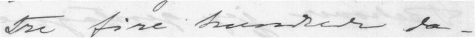Tre fire hundrede da-
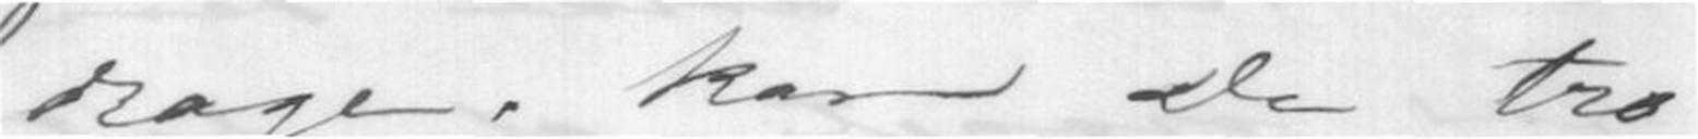dage, kan De tro,
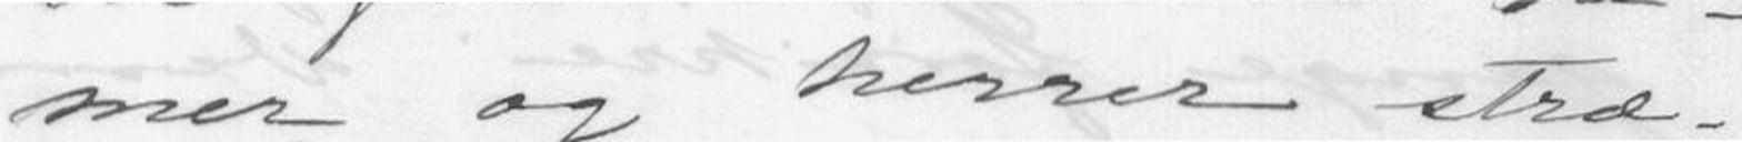mer og herrer stræ-
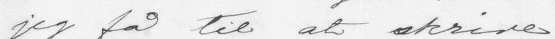jeg få til at skrive
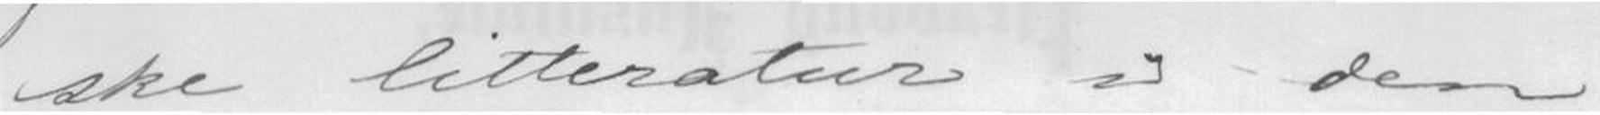ske litteratur i den
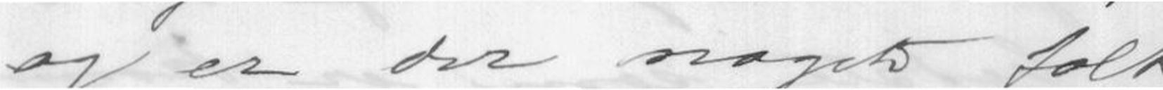og er der noget folk
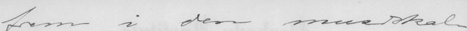frem i den musikal-
